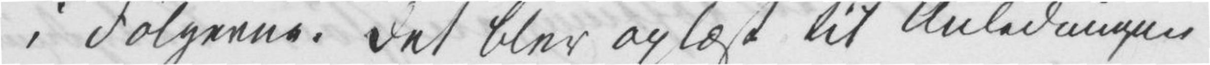i Følgerne. Det blev oplæst til Anledningen
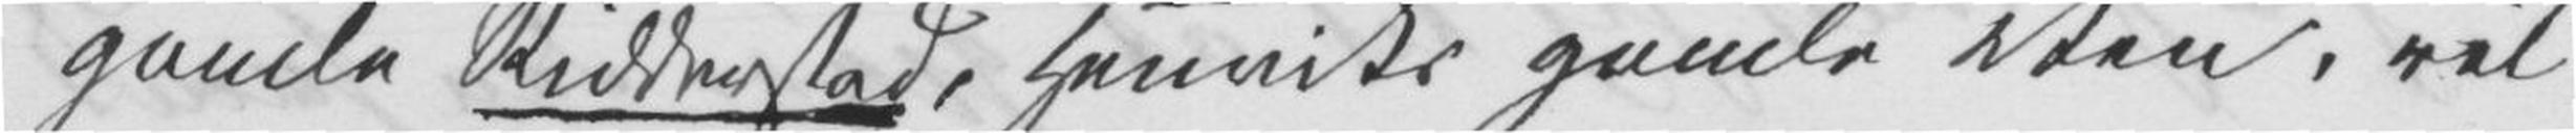gamle Ridderstad, Henriks gamle Ven, vil
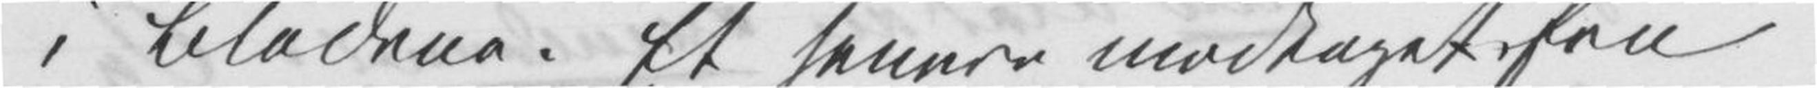i Bladene. Et senere modtaget fra
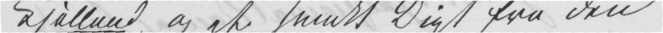Kjelland og et smukt Digt fra den
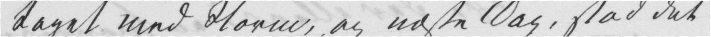taget med Storm, og næste Dag, stod det
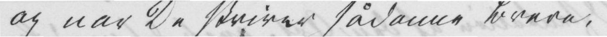og når De skriver sådanne Breve,
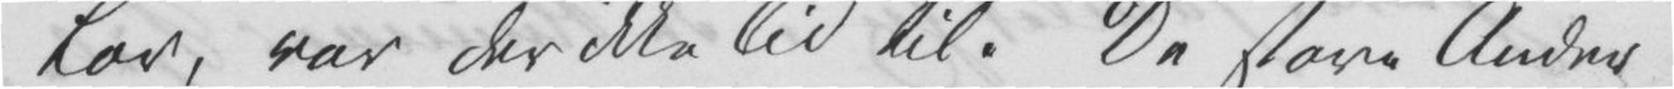Lov, var der ikke Tid til. De store Ånder
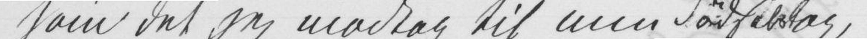som det jeg modtog til min Fødselsdag,
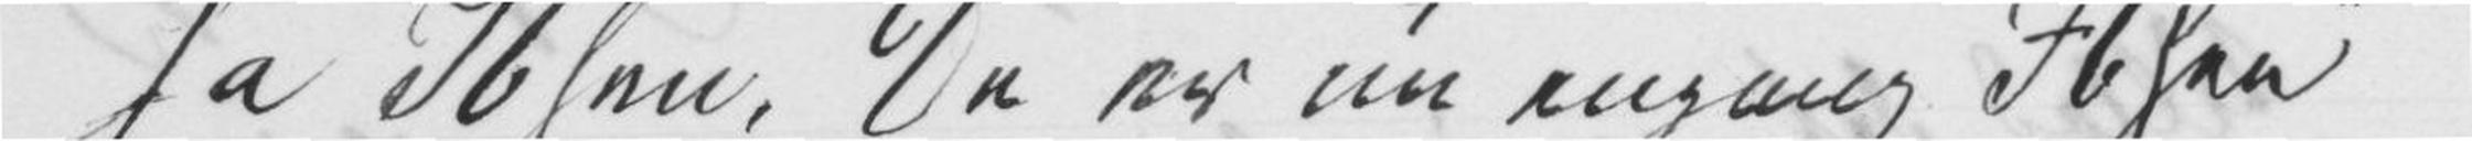Ja Ibsen, De er nu engang «Ibsen»
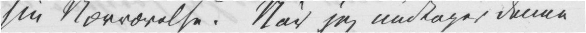sin Nærværelse. Når jeg undtager denne
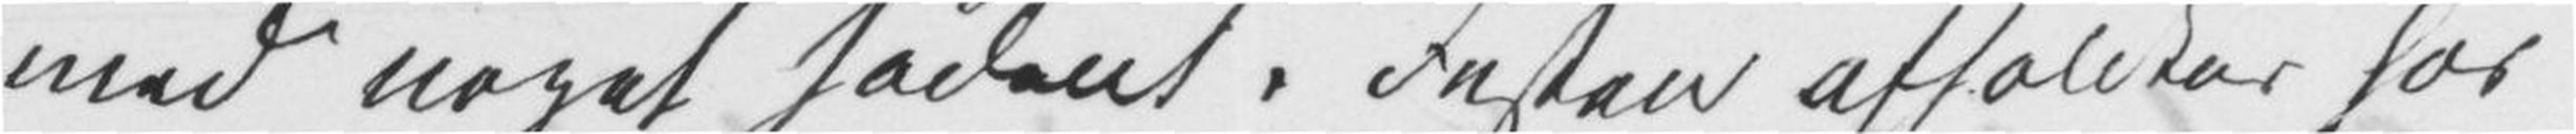med noget sådant. Festen afholdtes hos
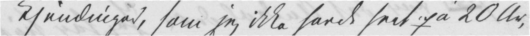Kjendinger, som jeg ikke havde seet på 20 År,
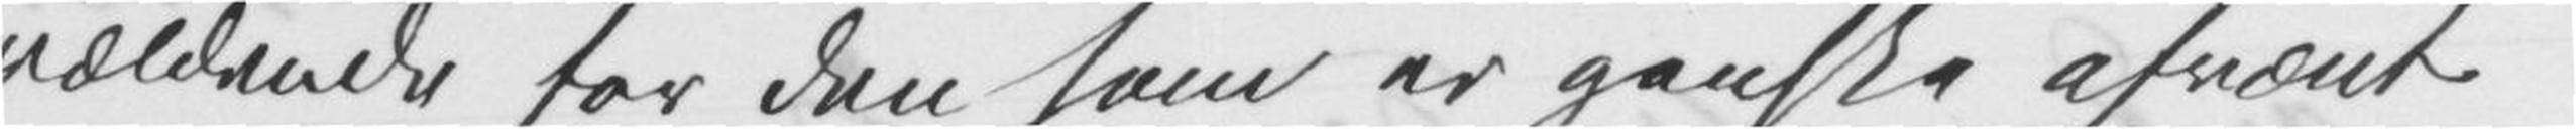vældende for den som er ganske afvænt
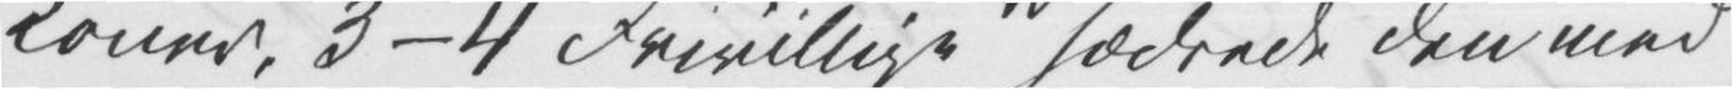Koner, 3 – 4 Frivillige hædrede den med
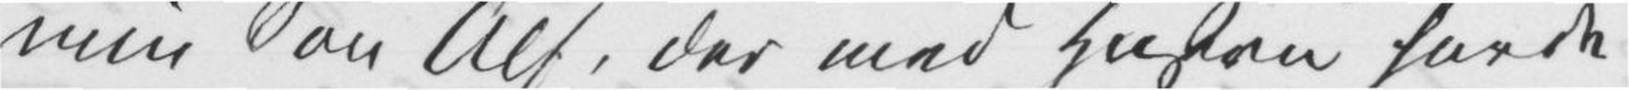min Søn Alf, der med Hustru havde
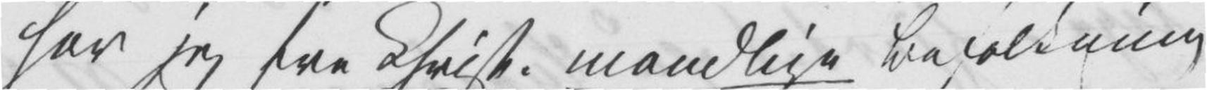har jeg fra Christ. mandlige Befolkning
